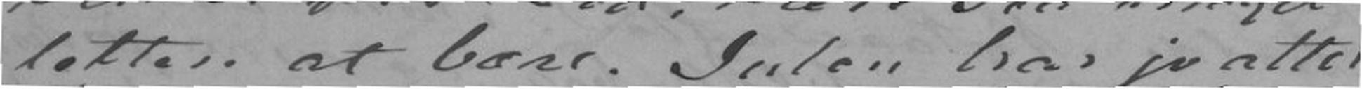lettere at bære. Julen har jo atter
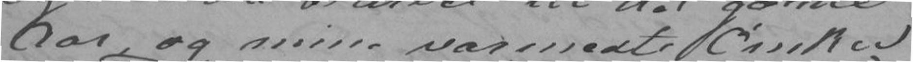Aar, og mine varmeste Ønsker
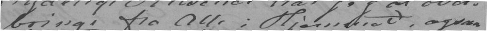bringe fra Alle i Hjemmed, ogsaa
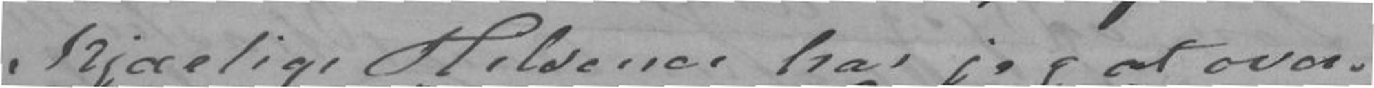Kjærlige Hilsener har jeg at over-
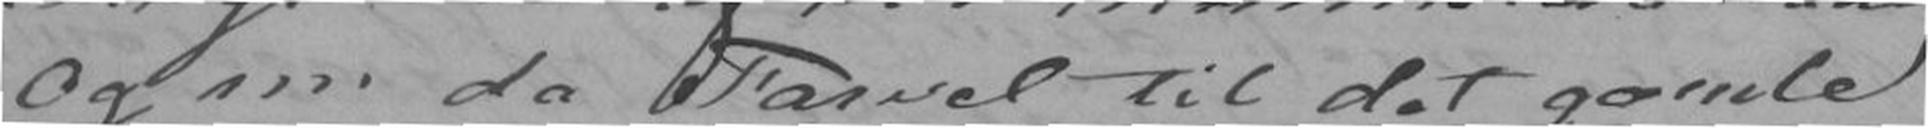Og nu da Farvel til det Gamle
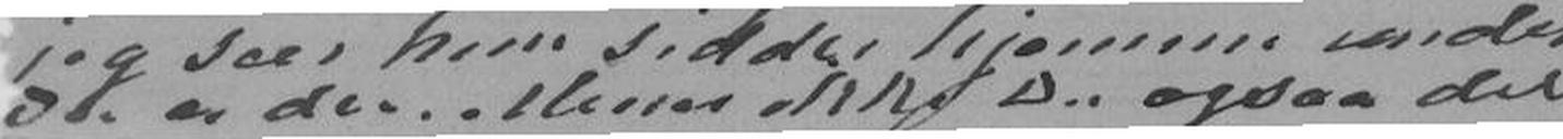Du er der. Mener ikke Du ogsaa det
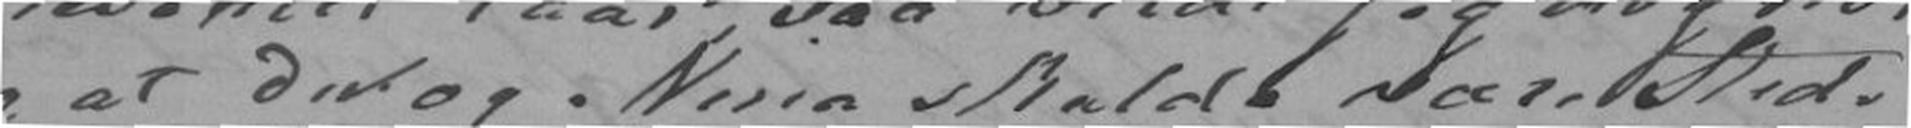at din og Mina skuld være Sted-
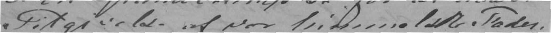Tilgivelse af vor himmelske Fader.
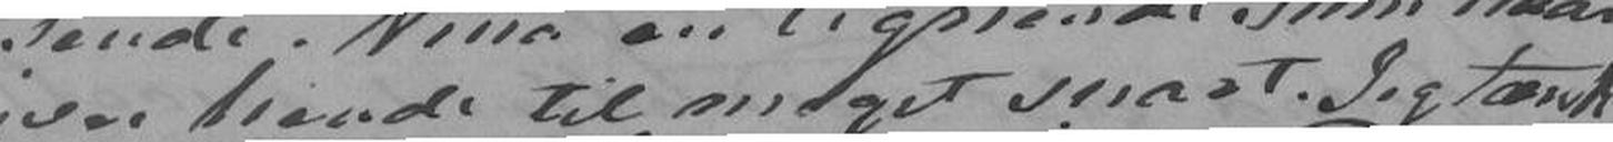iver havde til meget snart. Jeg tænkte
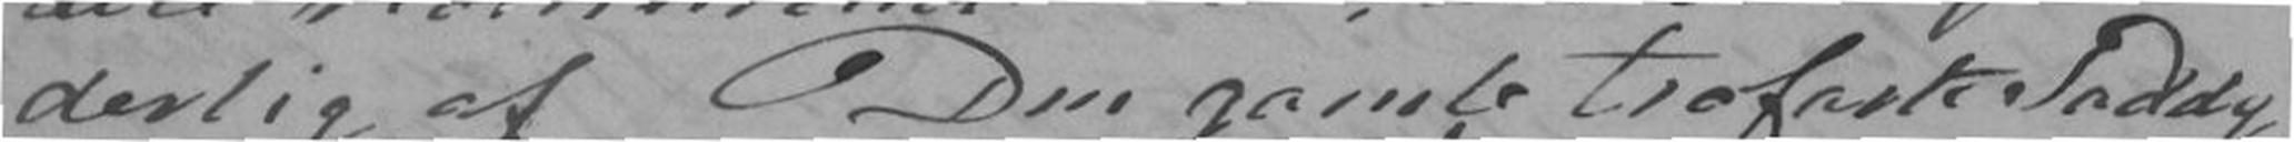derlig af Din gamle trofaste Paddy
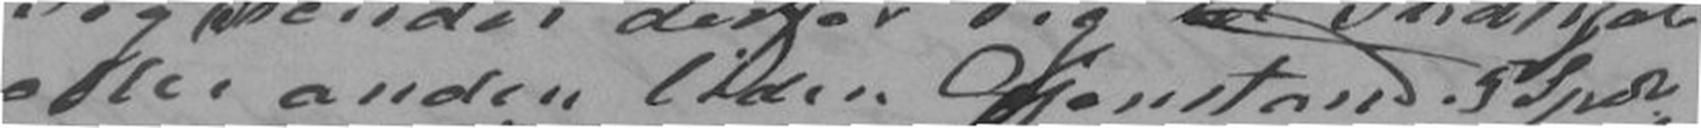eller anden liden Gjenstand 5 Spd.
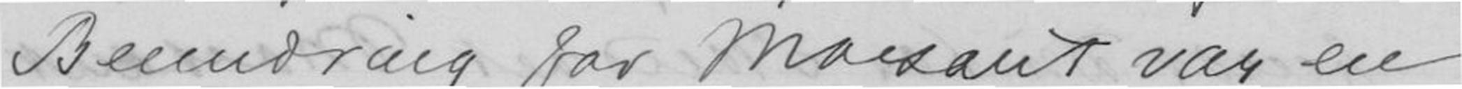Beundring for Mozart var en
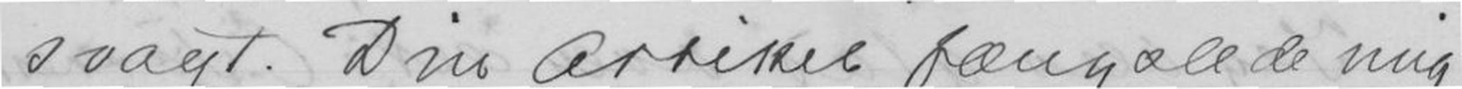svagt. Din Artikel fængænde mig
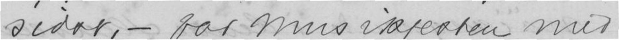sidst,- for Musikfesten med
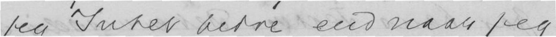jeg Intet bedre end naar jeg
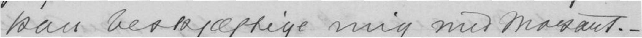kan beskjæptige mig med Mozart.-
